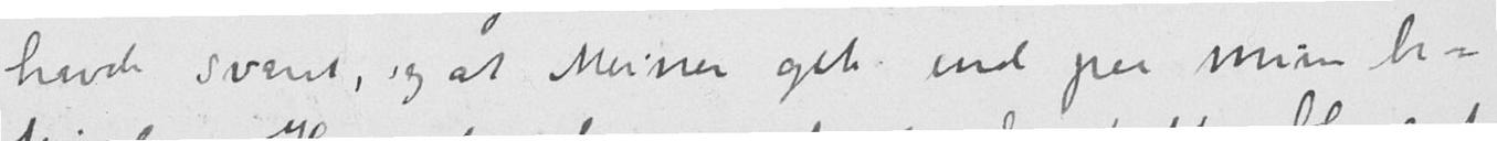havde svaret, og at Meiner gik ind paa mine be-
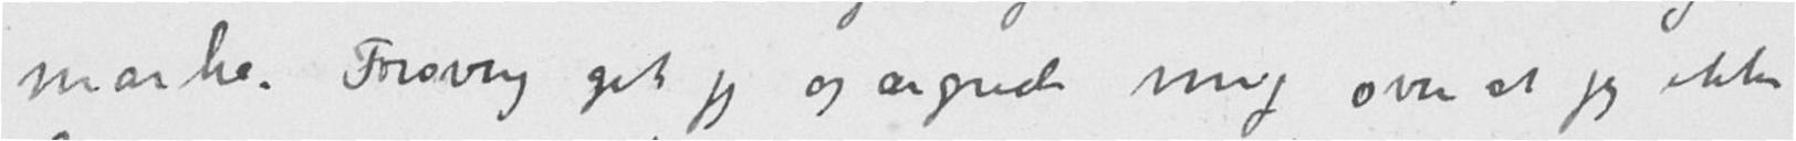marke. Forøvrig gik jeg og ærgrede mig over at jeg ikke
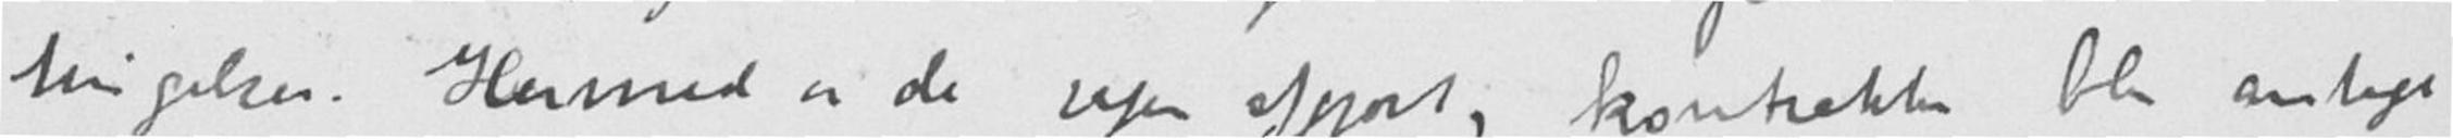tingelser. Hermed er da sagen afgjort, kontrakten blir antage-
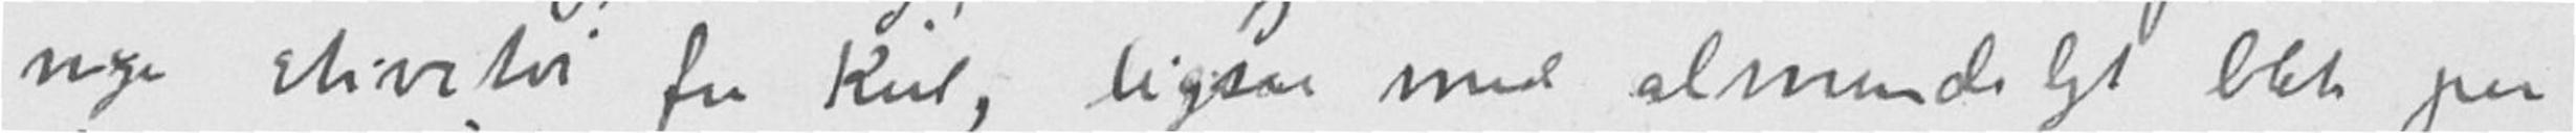nye stive tøi fra Kiel, ligesaa med almindeligt blæk paa
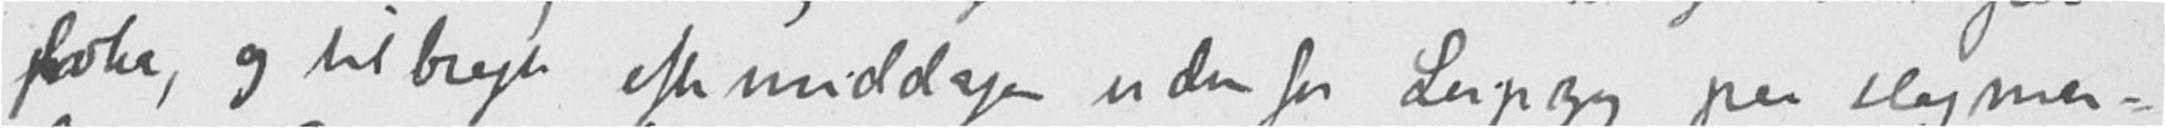boka, og tilbragte eftermiddagen udenfor Leipzig paa slagmar-
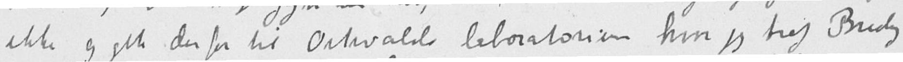ikke og gik derfor til Ostwalds laboratorium hvor jeg traf Bredig
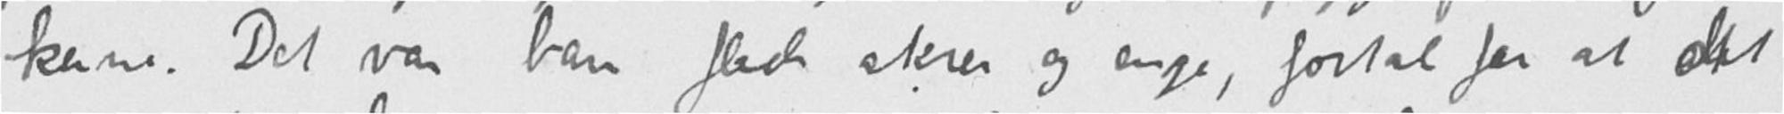kene. Det var bare flade akrer og enge, fortæl for at det
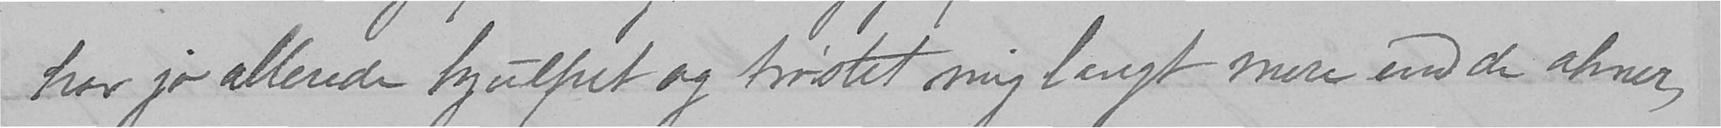har jo allerede hjulpet og trøstet mig langt mere end de ahner,
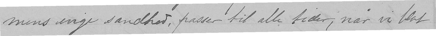mens evige sandhed, passer til alle tider, når vi blot
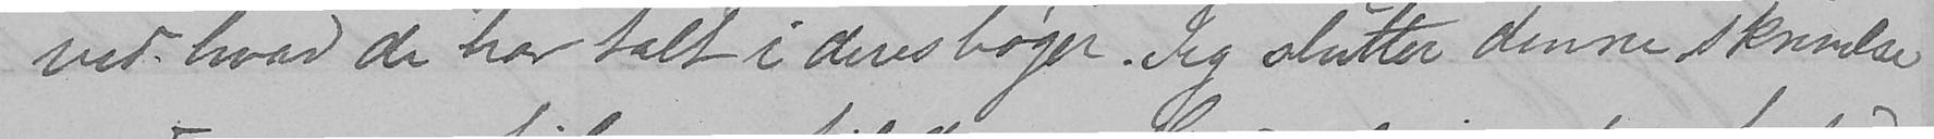ved hvad de har talt i deres bøger. Jeg slutter denne skrivelse
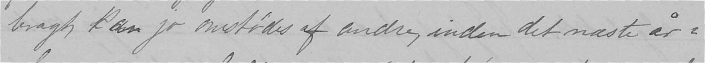bragt, kan jo omstødes af andre, inden det næste år-
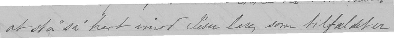at stå så tvert imod Jesu lære, som tilfældet er
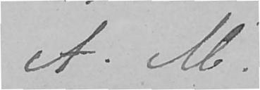A.M.
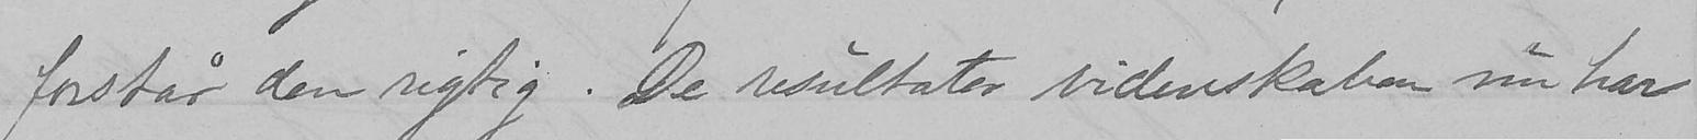forstår den rigtig. De resultater videnskaben nu har
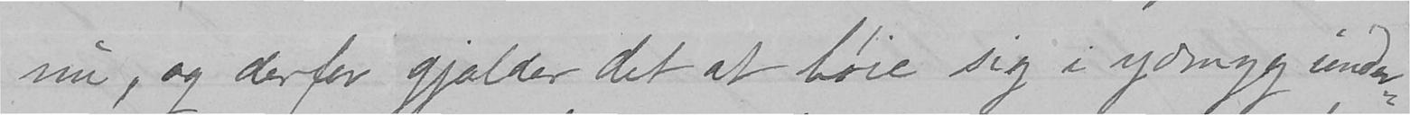nu, og derfor gjælder det at bøie sig i ydmyg under-
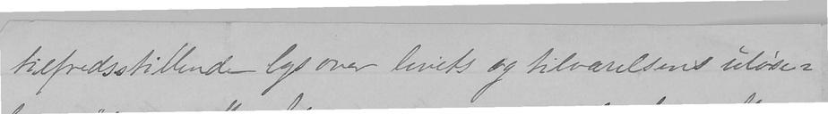tilfredsstillende lys over livets og tilværelsens uløse-
